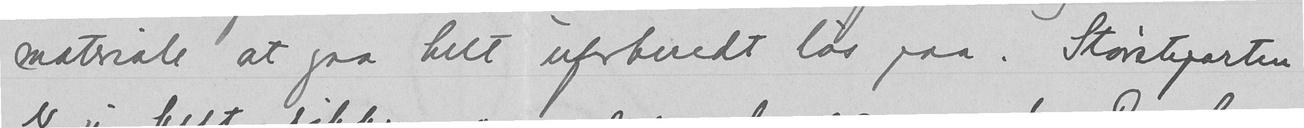materiale at gaa helt uforberedt løs paa. Størsteparten
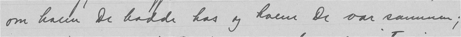om hvem De bodde hos og hvem De var sammen;
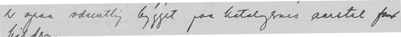er ogsaa væsentlig bygget paa katalogernes aarstal for
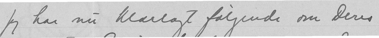Jeg har nu klarlagt følgende om Deres
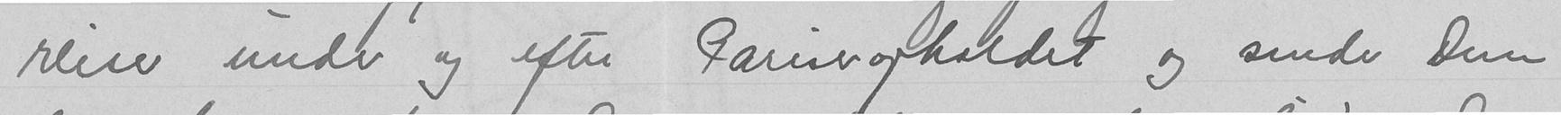reise under og efter Pariseropholdet og sender Dem
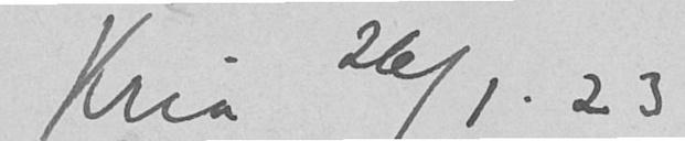Kria 26/1 - 23
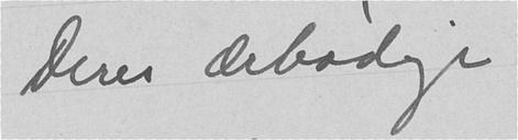Deres ærbødige
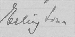Erling Lone.
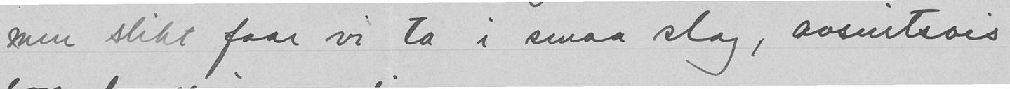men slikt faar vi ta i smaa slag, avsnitsvis
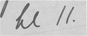kl 11.
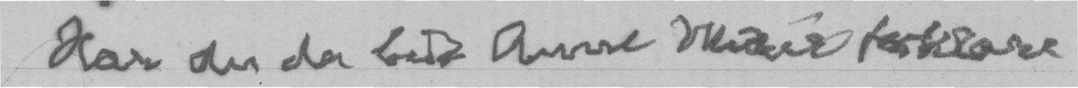Har du da bedt Anne Marie forklare
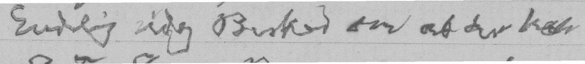Endelig idag Besked om at du har
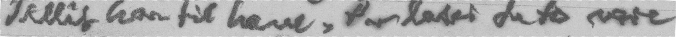Tillit har til henne. Du lader de to være
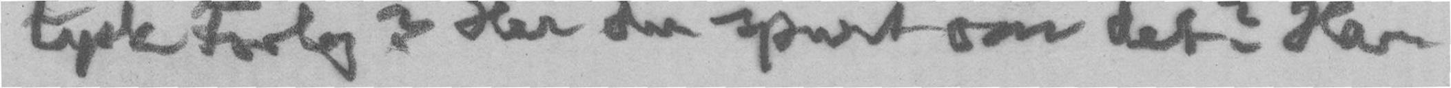tysk Forlag? Har du spurt om det? Har
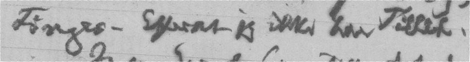Finger. Efterat jeg ikke har Tillit.
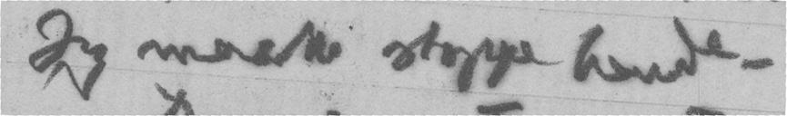Jeg maatte stoppe hende.
